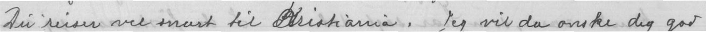Du reiser vel snart til Christiania. Jeg vil da ønske dig god
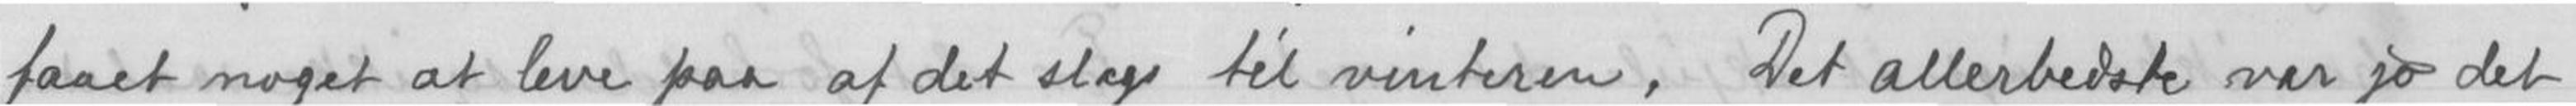faaet noget at leve paa af det slags til vinteren. Det allerbedste var jo
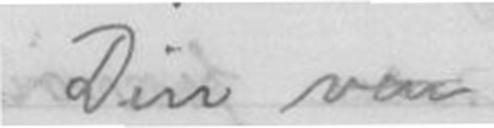Din ven
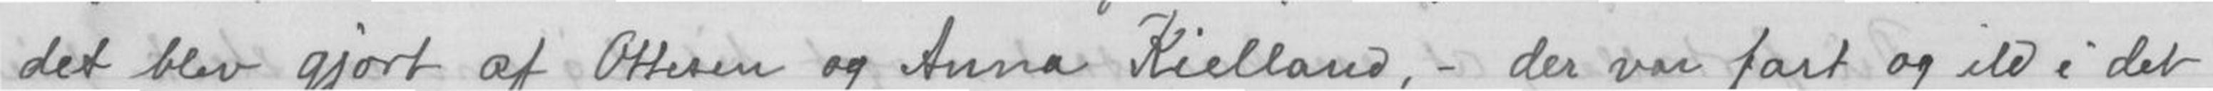det blev gjort af Ottesen og Anna Kielland, - der var fart og ild i det
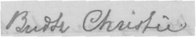Budtz Christie
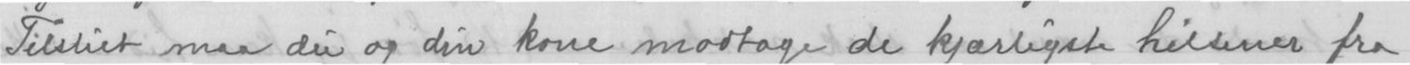Tilslut maa du og din kone modtage de kjærligste hilsener fra
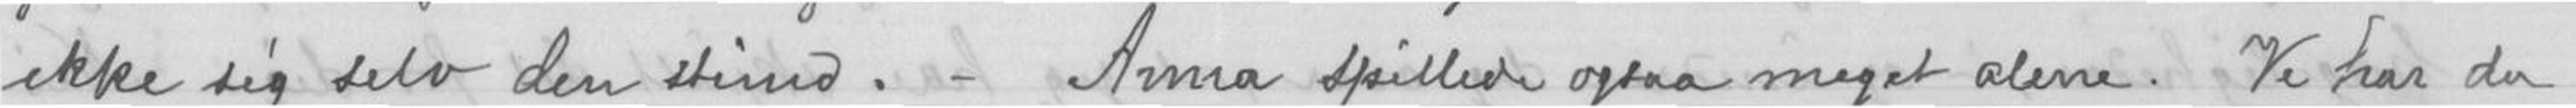ikke sig selv den stund. - Anna spillede ogsaa meget alene. Vi har da
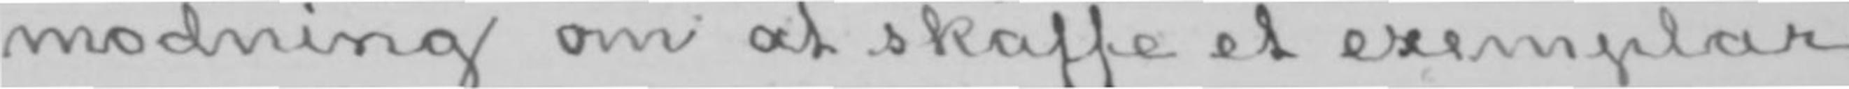modning om at skaffe et exemplar
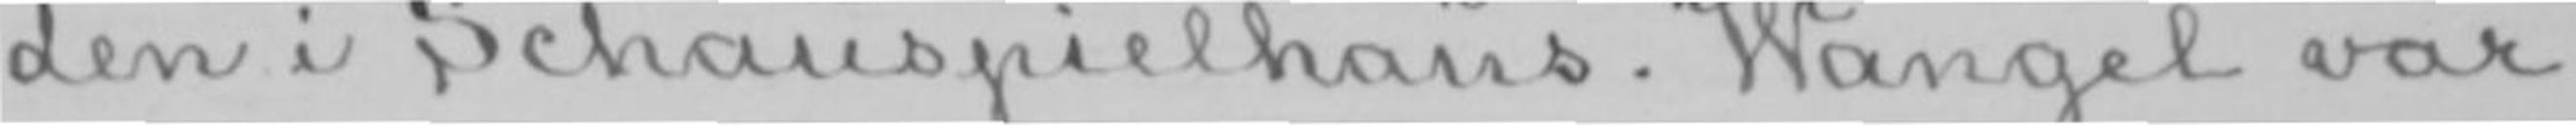den i Schauspielhaus. Wangel var
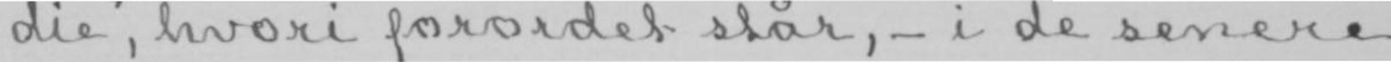die», hvori forordet står,- i de senere
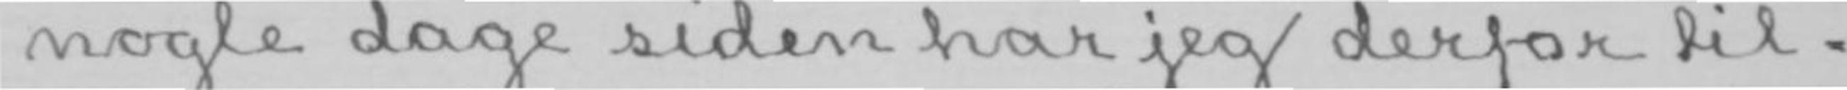nogle dage siden har jeg derfor til-
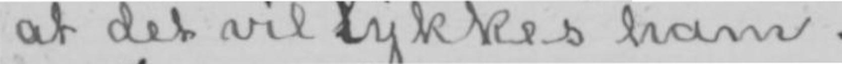at det vil lykkes ham.
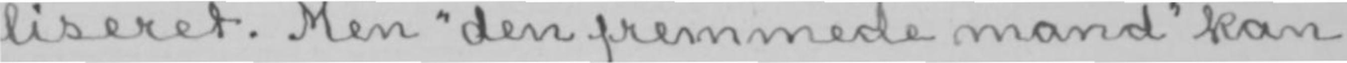liseret. Men «den fremmede mand» kan
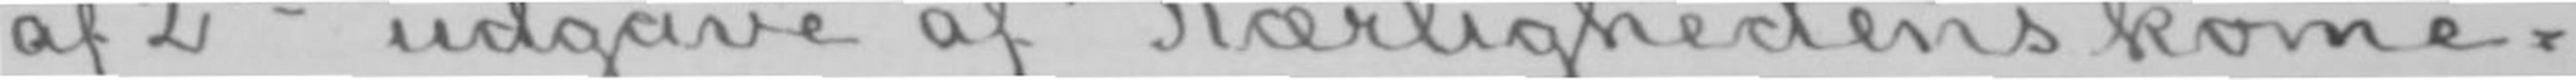af 2den udgave af «Kærlighedens kome-
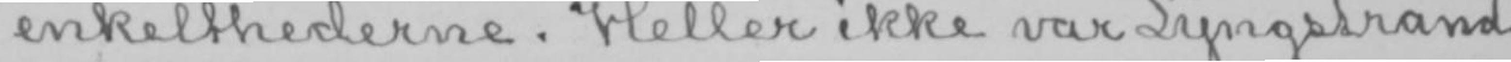enkelthederne. Heller ikke var Lyngstrand
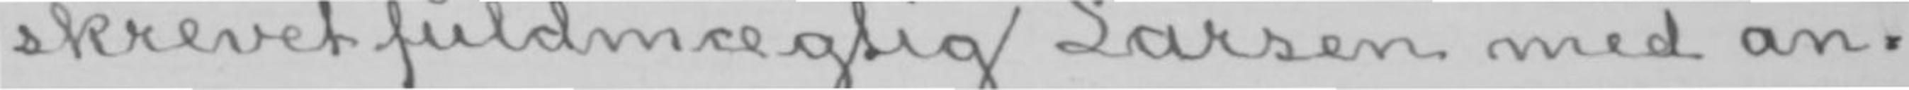skrevet fuldmægtig Larsen med an-
